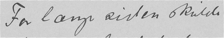For længe siden skulde
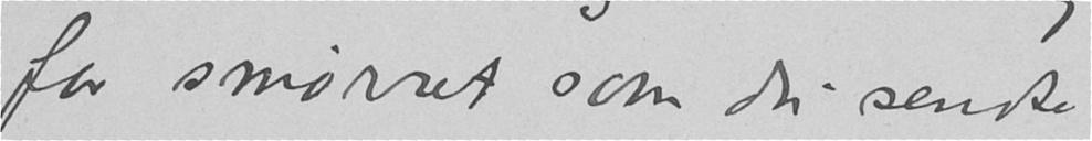for smørret som du sendte
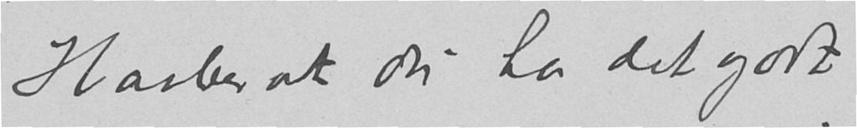Haaber at du har det godt
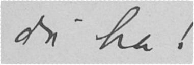du ha!
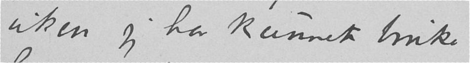uken jeg har kunnet bruke
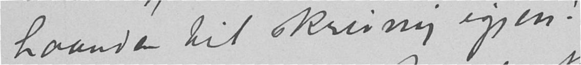haanden til skrivning igjen!
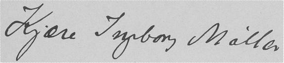Kjære Ingeborg Møller,
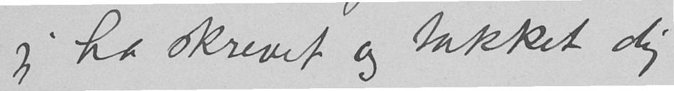jeg ha skrevet og takket dig
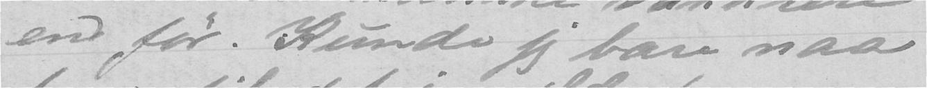end før. Kunde jeg bare naa
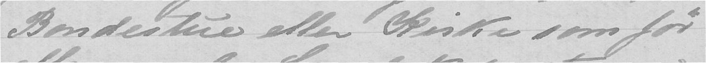Bondestue eller Kike som før
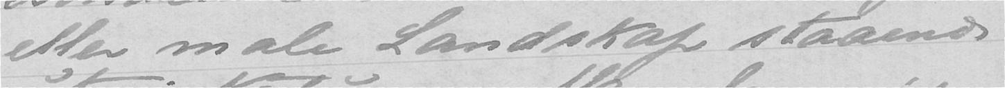eller male Landskap staaende
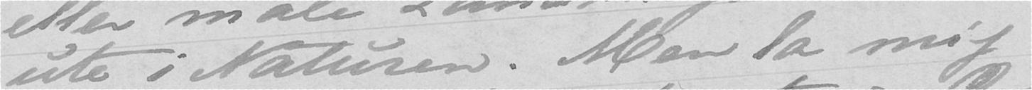ute i Naturen. Men la mig
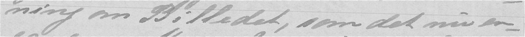ning om Billedet, som det nu en-
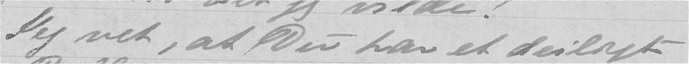Jeg vet, at Du har et deiligt
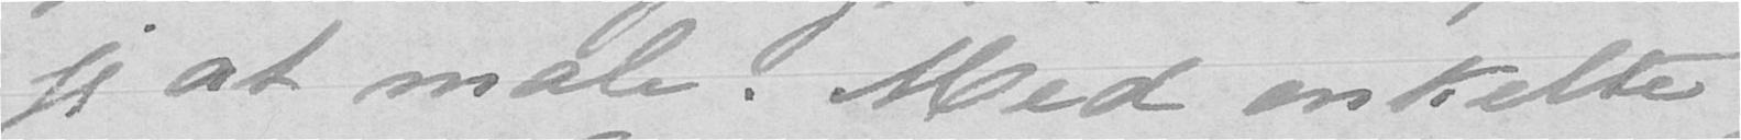jeg at male. Med enkelte
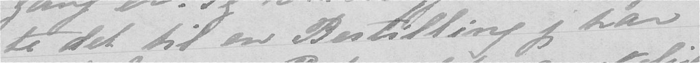te det til en Bestilling jeg har
